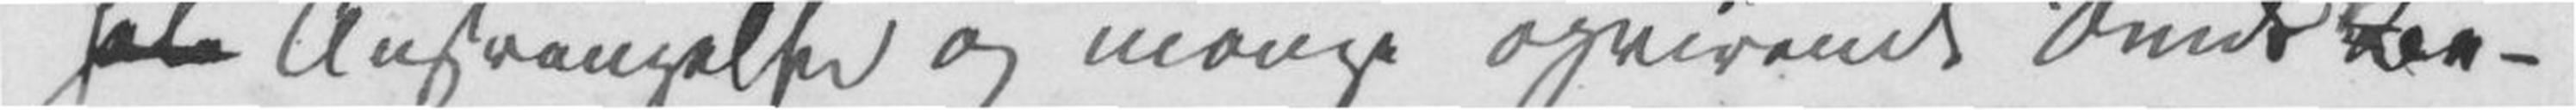hele Anstrengelse og mange oprivende Sindsbe¬
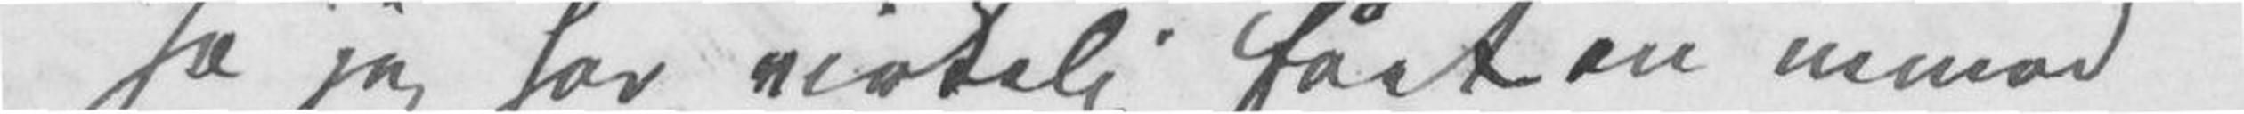Ja jeg har virkelig fået en uimod¬
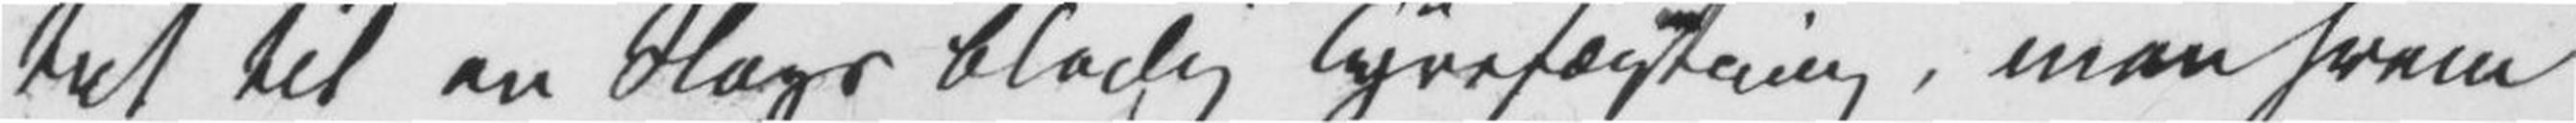tet til en Slags blodig Tyrefægtning, men hvem
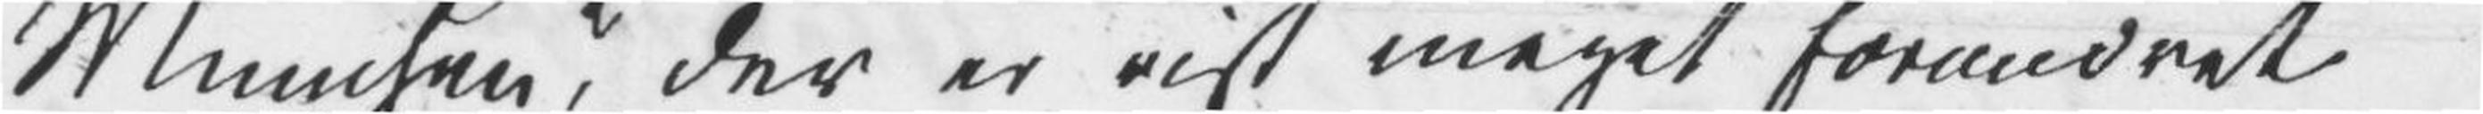München? Der er vist meget forandret
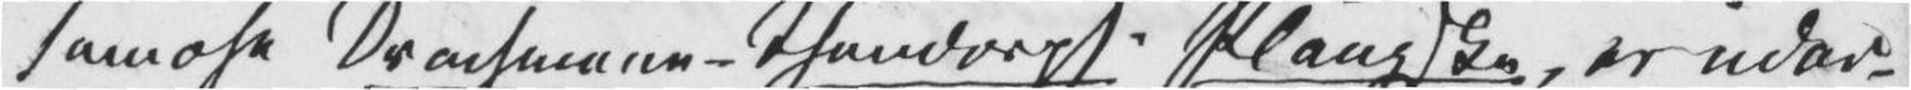famose Drachmann-Shandorph-Plougske, er udar¬
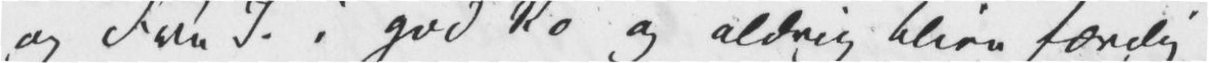og Fru I. i god Ro og aldrig blive færdig
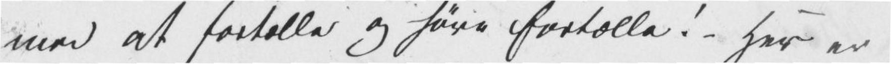med at fortælle og høre fortælle! – Her er
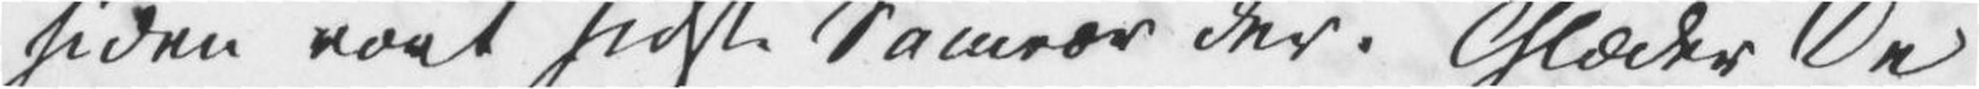siden vort sidste Samvær der. Glæder De
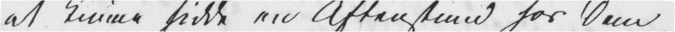at kunne sidde en Aftenstund hos Dem
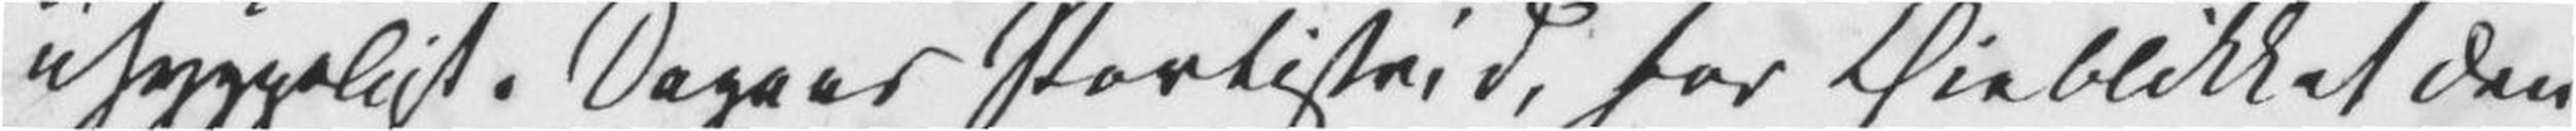uhyggeligt. Dagens Partistrid, for Øieblikket den
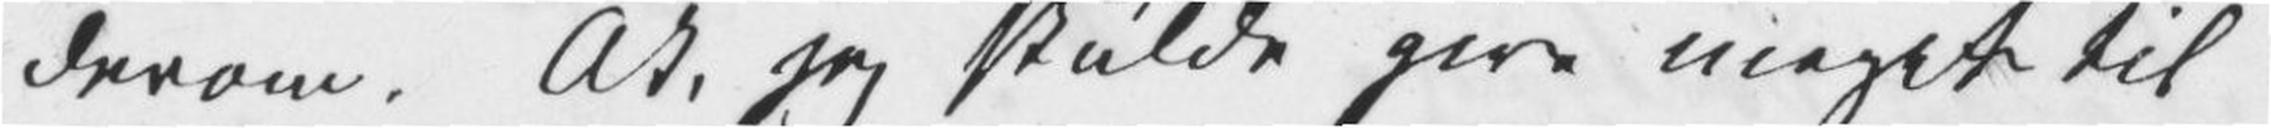derom. Ak, jeg skulde give meget til
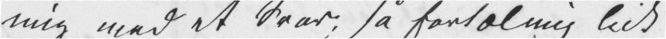mig med et Svar, så fortæl mig lidt
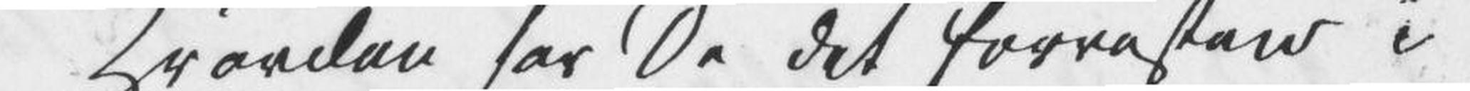Hvordan har De det forresten i
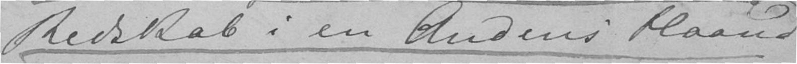Redskab i en Andens Haand
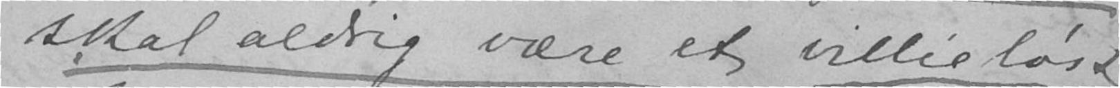skal aldrig være et villieløst
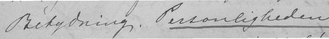Betydning. Personligheden
